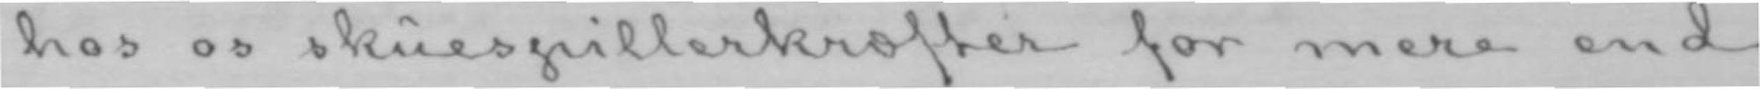hos os skuespillerkræfter for mere end
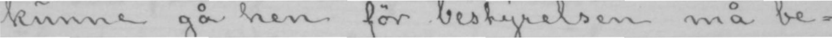kunne gå hen før bestyrelsen må be-
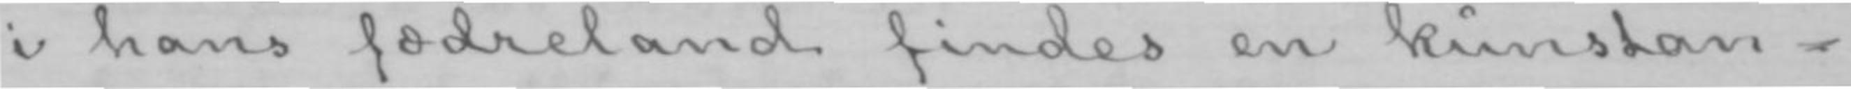i hans fædreland findes en kunstan-
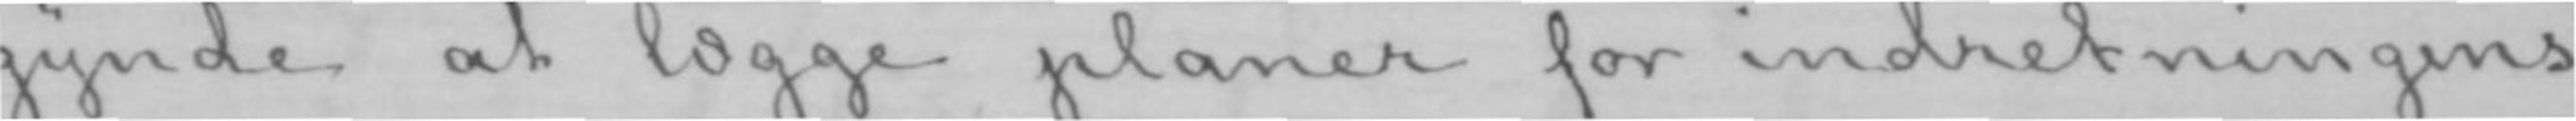gynde at lægge planer for indretningens
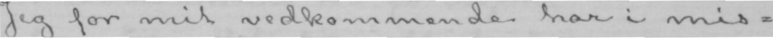Jeg for mit vedkommende har i mis-
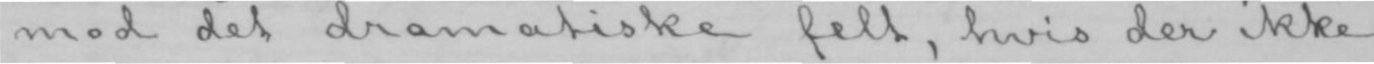mod det dramatiske felt, hvis der ikke
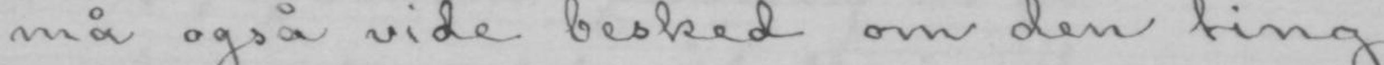må også vide besked om den ting.
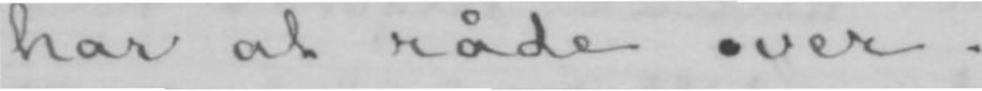har at råde over.
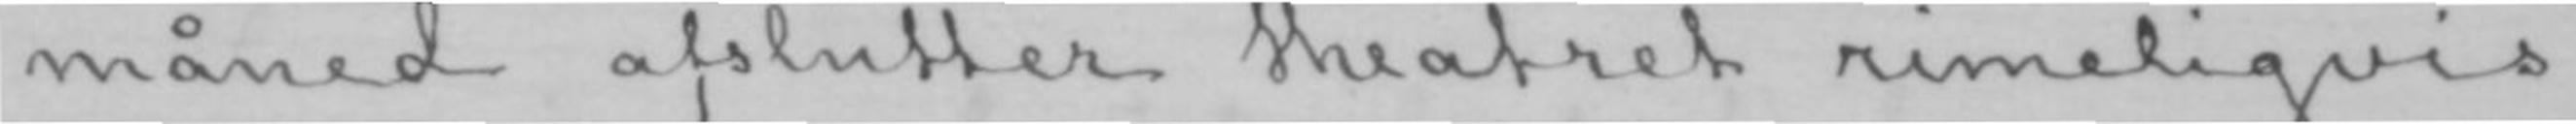måned afslutter theatret rimeligvis
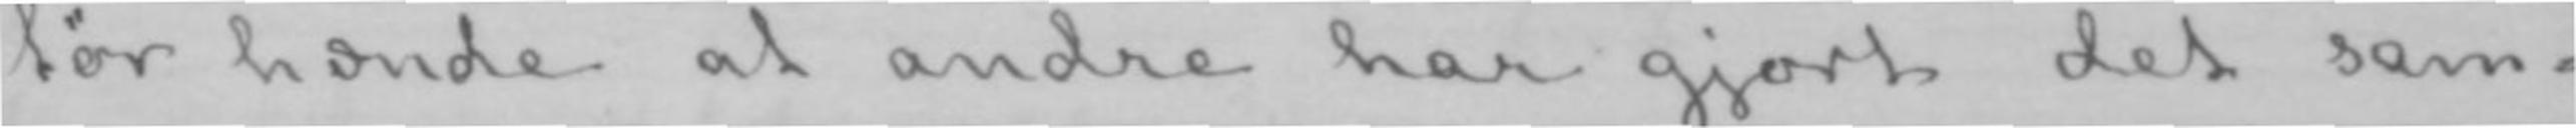tør hænde at andre har gjort det sam-
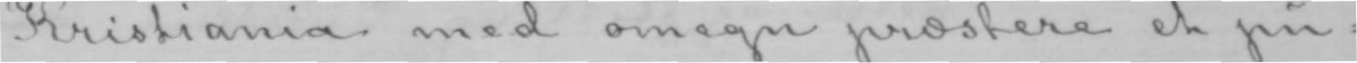Kristiania med omegn præstere et pu-
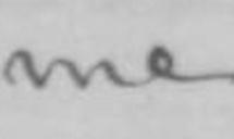me.
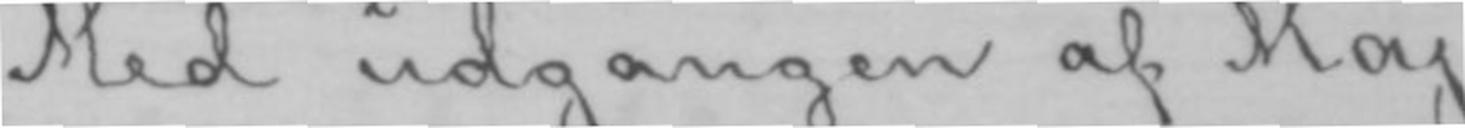Med udgangen af Maj
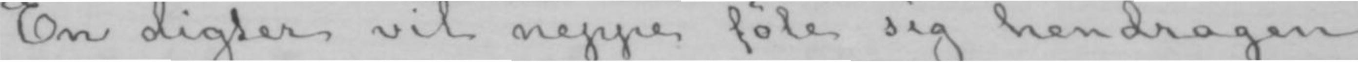En digter vil neppe føle sig hendragen
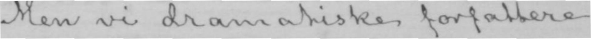Men vi dramatiske forfattere
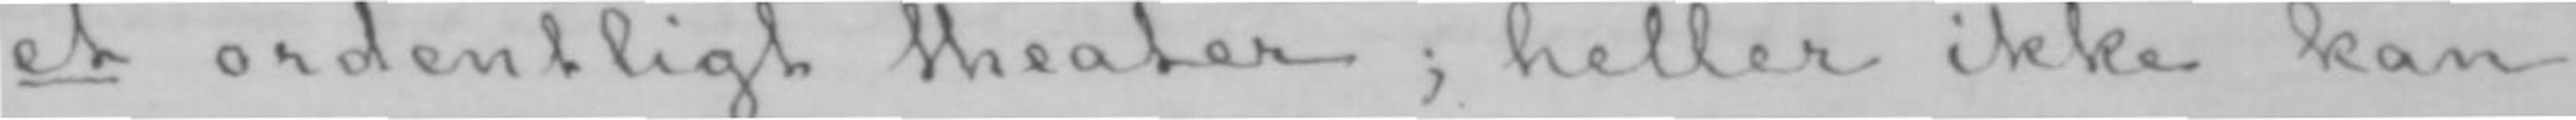et ordentligt theater; heller ikke kan
